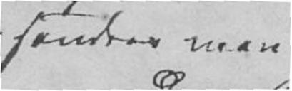fordrer man
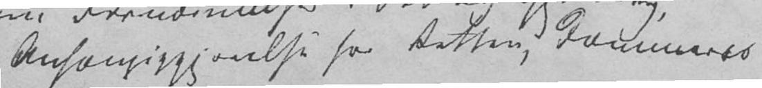Avhængiggjørelse for Retten, Dommeres
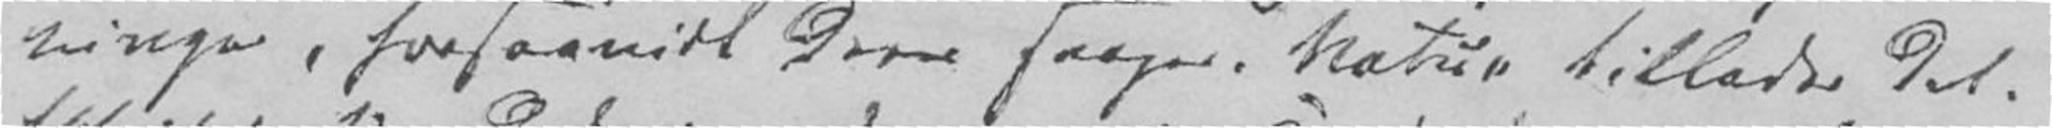ninger, forsaavidt deres svagere Natur tillader det.
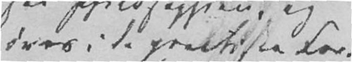øves i de practiske For-
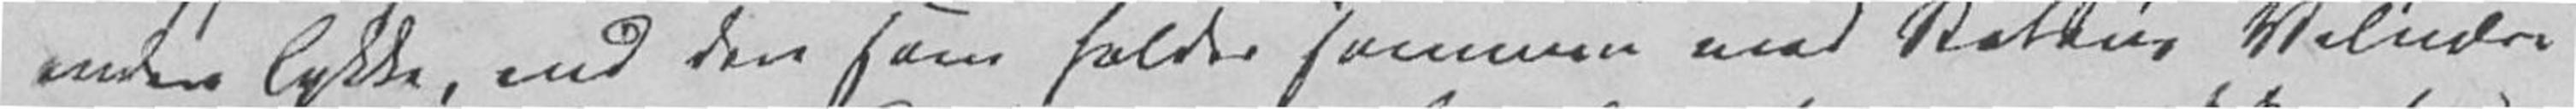anden Lykke, end den som falder sammen med Statens Velvære
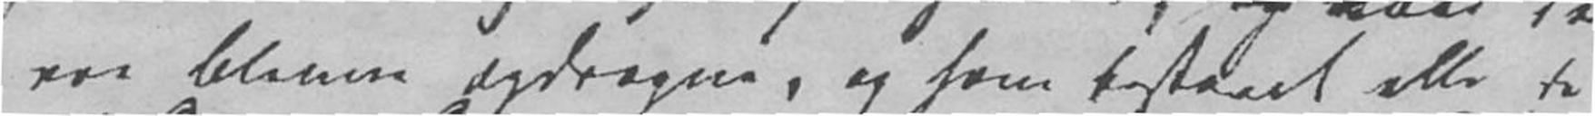ere blevne opdragne, og have bestaaet alle de
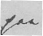paa
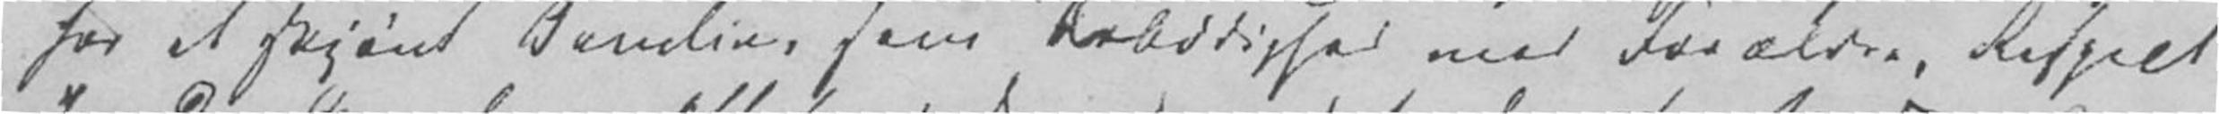for et skjønt Samliv, som Ærbødighed mod Forældre, Respect
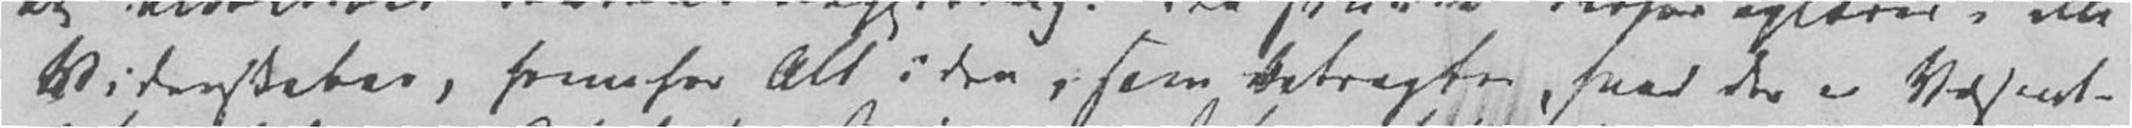Videnskaber, fremfor Alt i dem, som betragter, hvad der er Væsent-
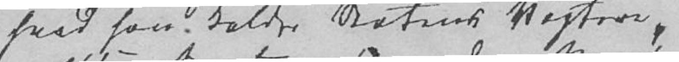hvad han kalder Statens Vogtere.
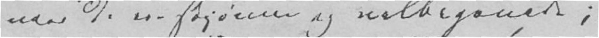naar de ere skjønne og velbegavede;
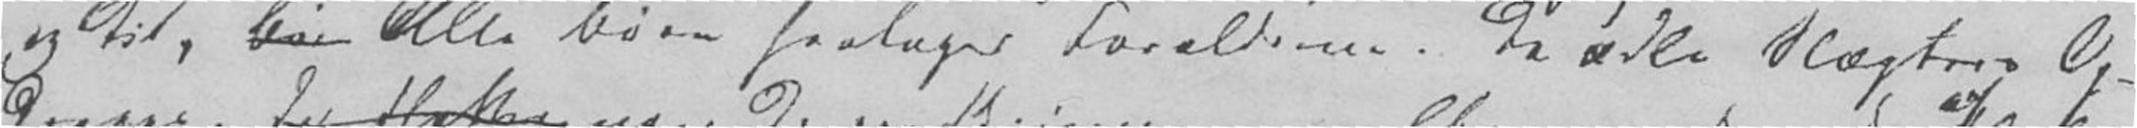og Dit. Bør Alle Børn fratages Forældrene. De ædle Slægters op-
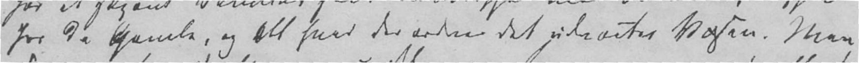for de Gamle, og Alt hvad der ordner det udvortes Væsen. Man
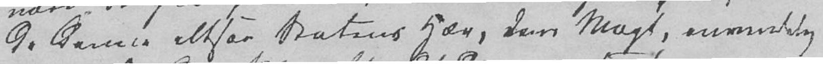De danne altsaa Statens Hær, dens Magt, anvendelig
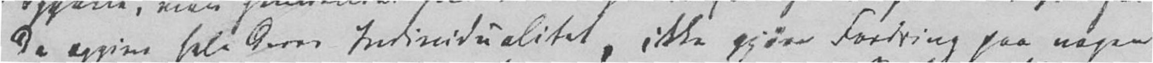de opgive hele deres Individualitet, ikke gjøre Fordring paa nogen
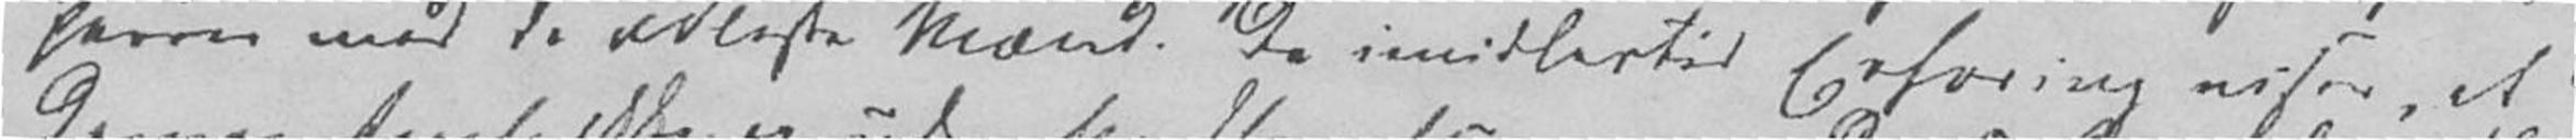parres med de ædleste Mænd. Da imidlertid Erfaring viser, at
